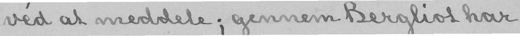véd at meddele; gennem Bergliot har
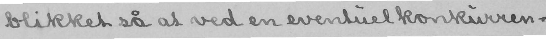blikket så at ved en eventuel konkurren-
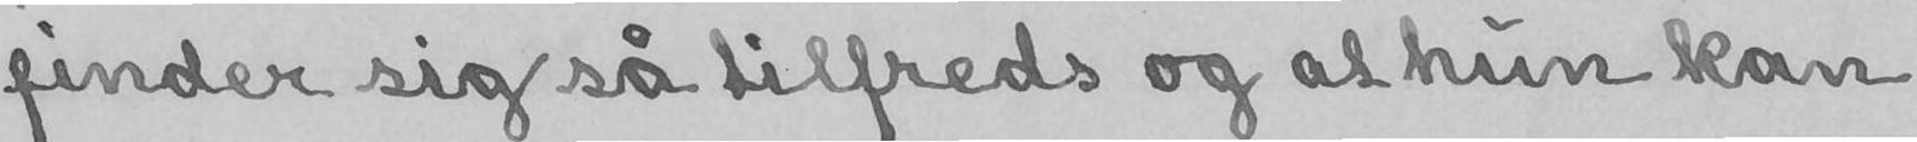finder sig så tilfreds og at hun kan
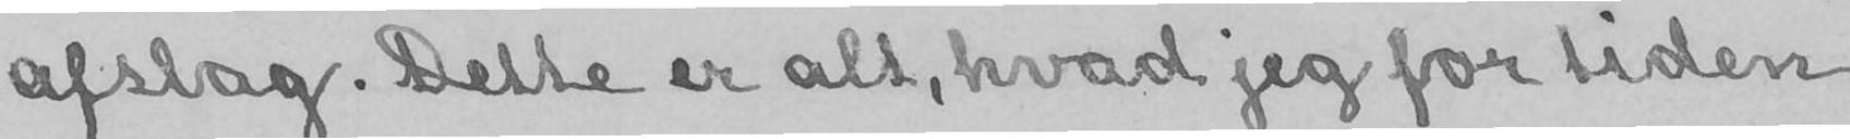afslag. Dette er alt, hvad jeg for tiden
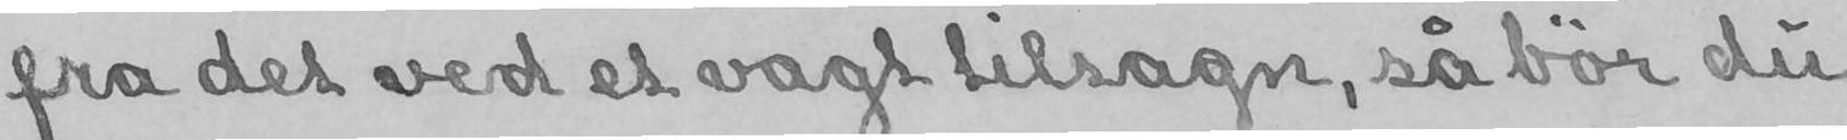fra det ved et vagt tilsagn, så bør du
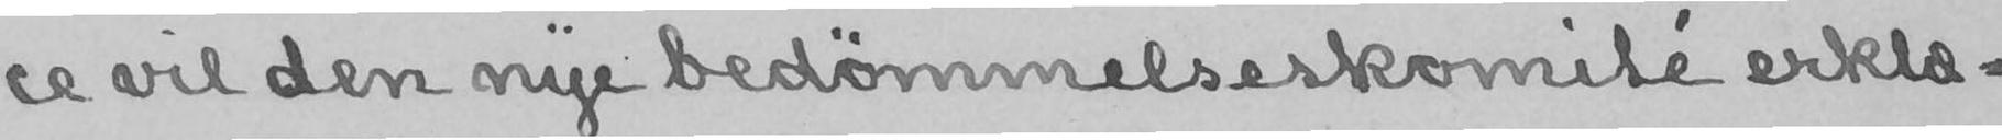ce vil den nye bedømmelseskomité erklæ-
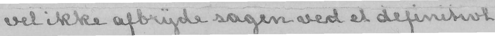vel ikke afbryde sagen ved et definitivt
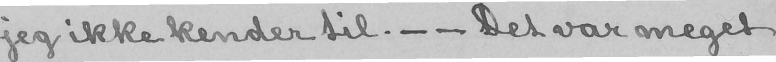jeg ikke kender til.-- Det var meget
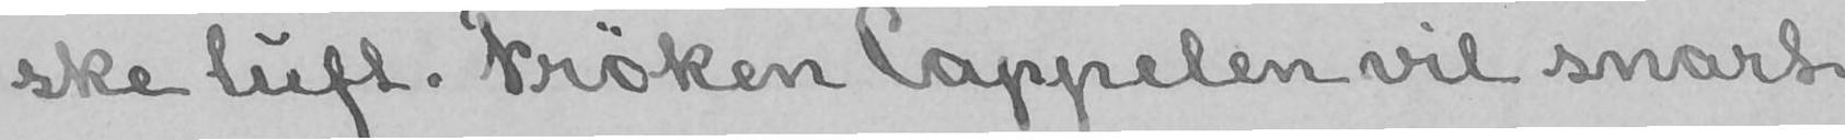ske luft. Frøken Cappelen vil snart
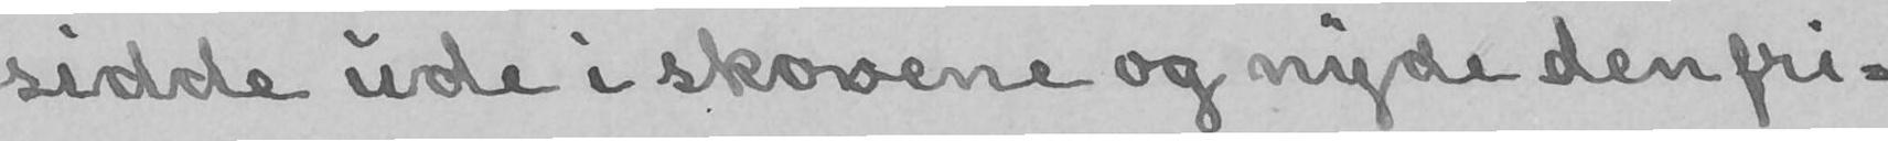sidde ude i skovene og nyde den fri-
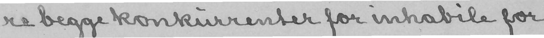re begge konkurrenter for inhabile for
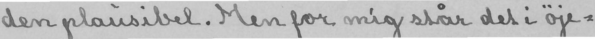den plausibel. Men for mig står det i øje-
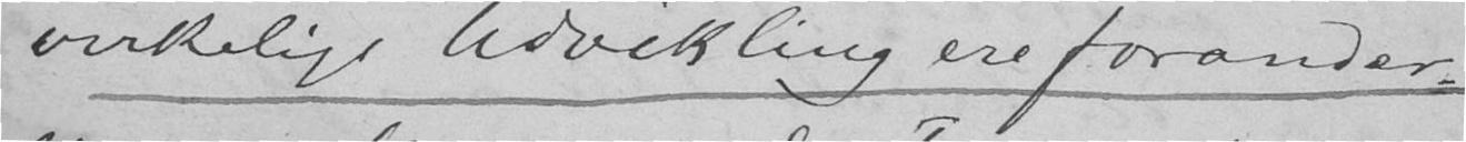virkelige Udvikling ere forander-
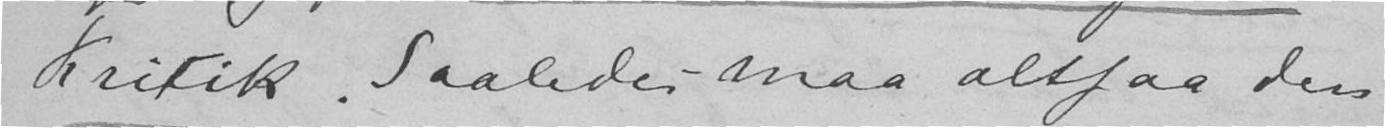Kritik. Saaledes maa altsaa den
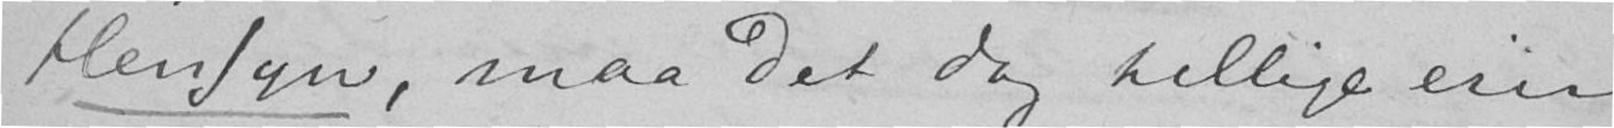Hensyn, maa det dog tillige erin-
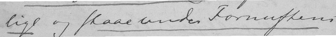lige og staae under Fornuftens
